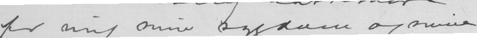for mig min sygdom og mine
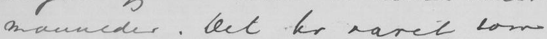maaneder. Det har været som
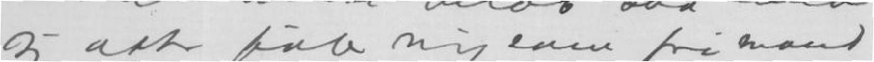jeg atter føle mig som fra mand
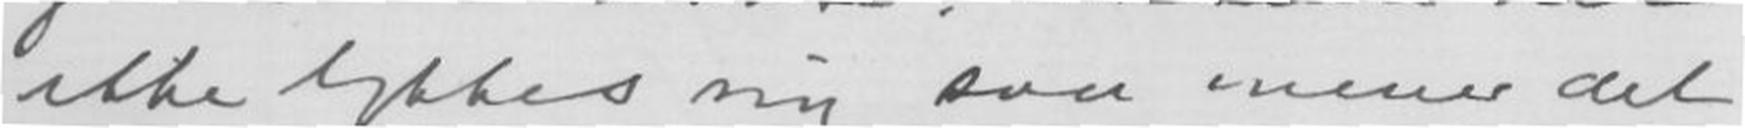ikke lykkes mig saa mener det
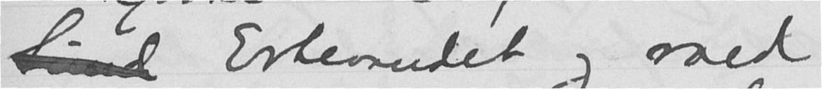Sund Ertevandet og roed
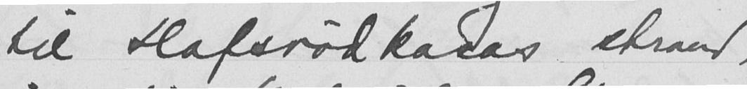til Hafsrødkasas strand,
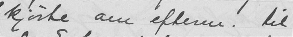kjørte om efterm. til
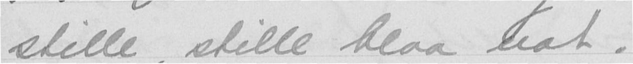stille, stille blaa nat.
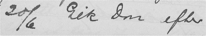20/6 Gik Don efter
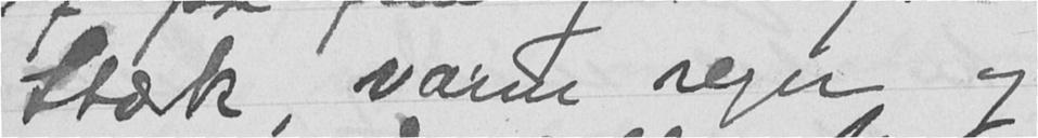Stærk, varm regn og
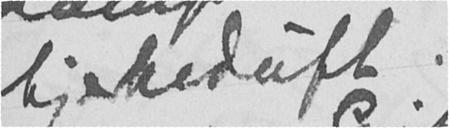bjærkeduft.
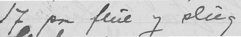17 paa flue og slug.
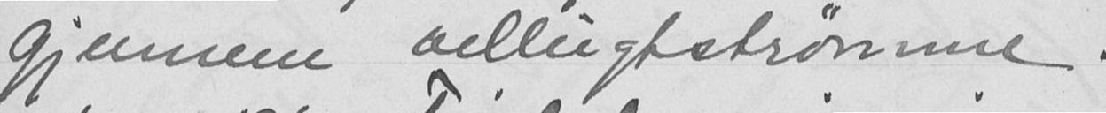gjennem vellugtstrømme.
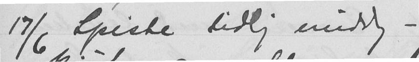17/6 Spiste tidlig middag -
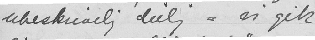ubeskrivelig deilig - vi gik
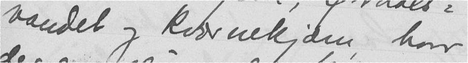vandet og Kværnekjern, hvor
